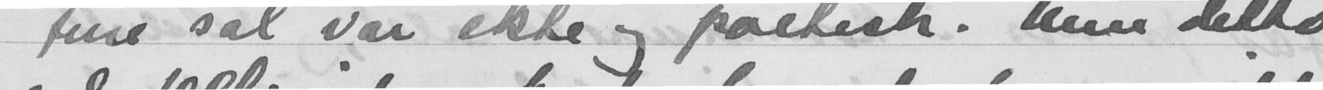fine sal var ekte og poltisk. Blev ditto
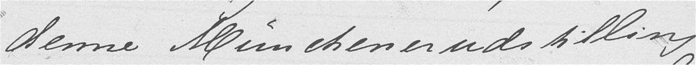denne Münchenerudstilling
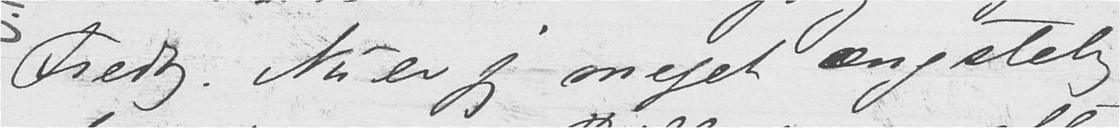Fredag. Nu er jeg meget ængstelig
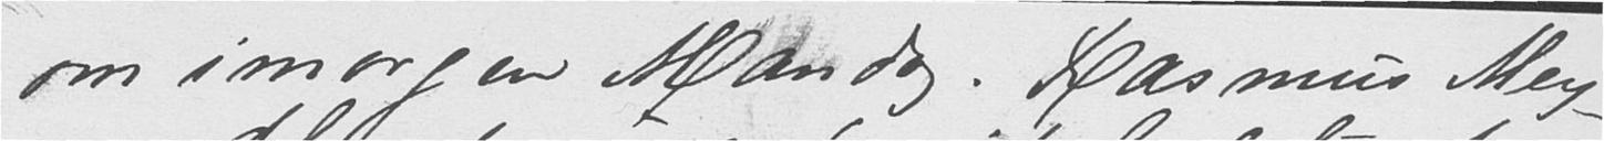om imorgen Mandag. Rasmus Mey-
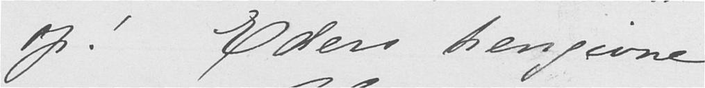op. Eders hengivne
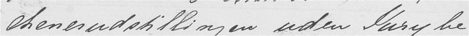chenerudstillingen uden Jury be-
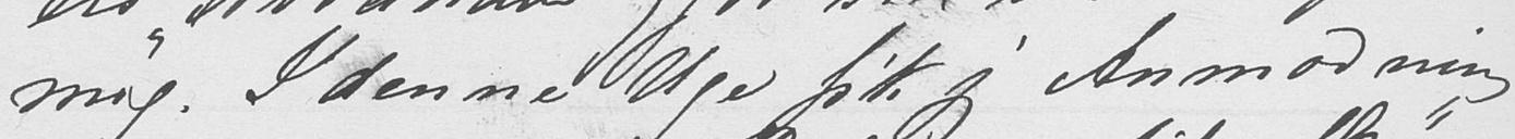mig. I denne Uge fik jeg Anmodmin
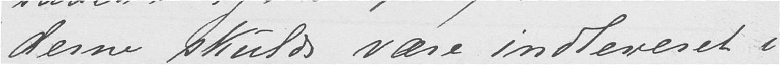derne skulde være indleveret i
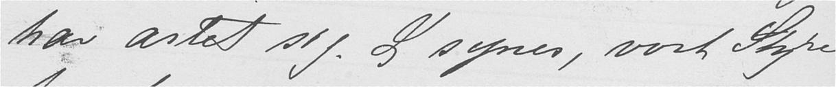har artet sig. Jeg synes, vort Styre
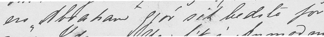ers Abraham gjør set bedste for
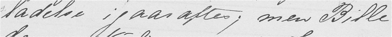ladelse igaaraftes; men Bille-
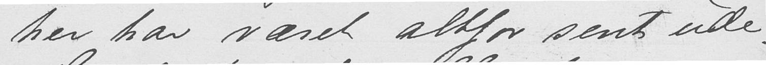her har været altfor sent ude.
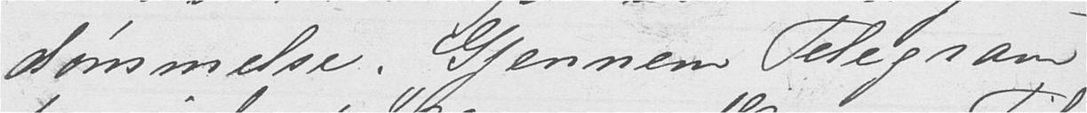dømmelse. Gjennem Telegram
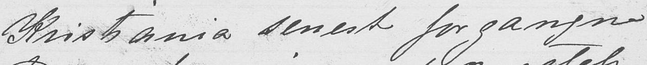Kristiania senest forgangne
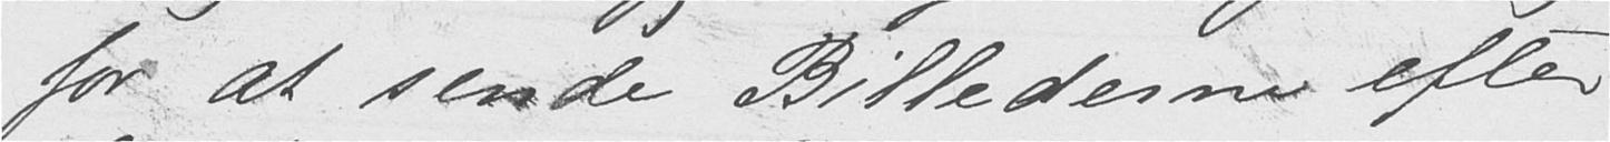for at sende Billederne efter
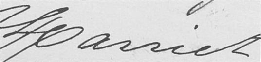Harriet
